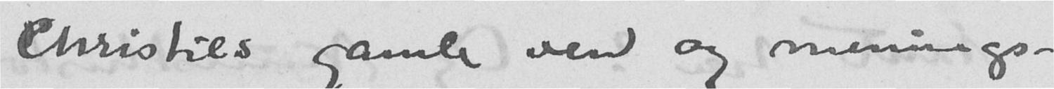Christies gamle ven og menings-
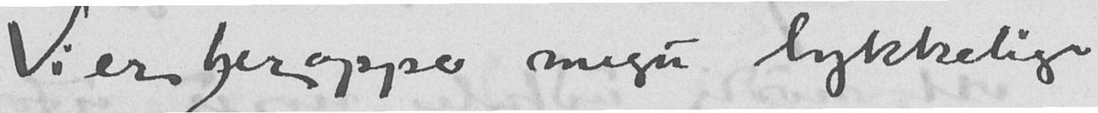Vi er her oppe mye lykkelige
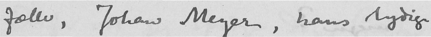fælle, Johan Meyer, hans lydige
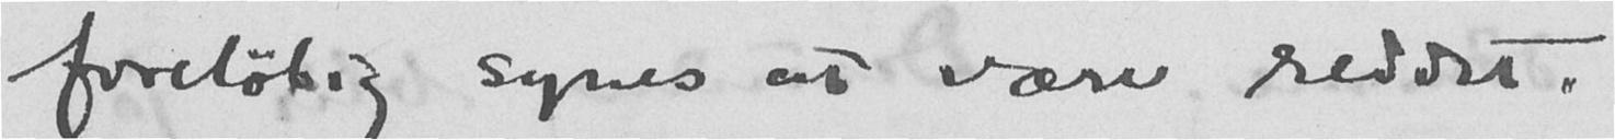foreløbig synes at være reddet.
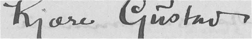Kjære Gustav
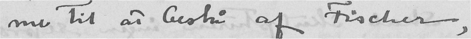me til at bestå af Fischer,
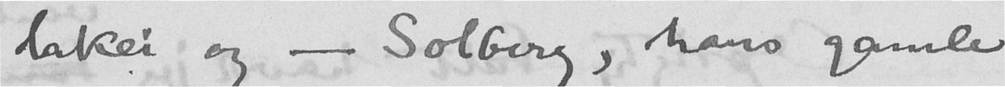lakei og - Solberg, hans gamle
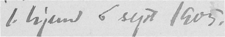T. hjem 6 sept. 1905.
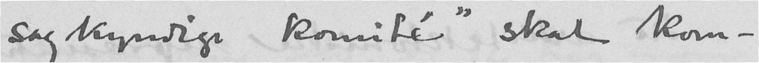sagkyndige komité" skal kom-
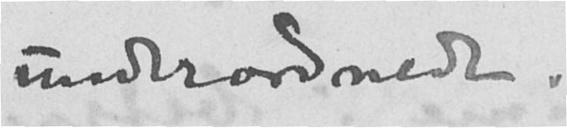underordnede.
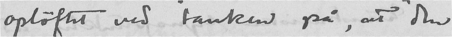opløftet ved tanken på, at "den
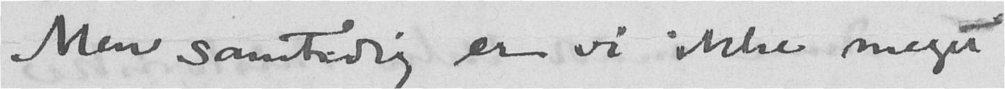Men samtidig er vi ikke meget
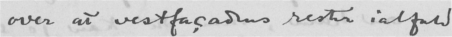over at vestfacadens rester ialfald
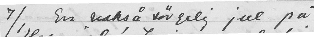7/1 En nokså sørgelig jul på
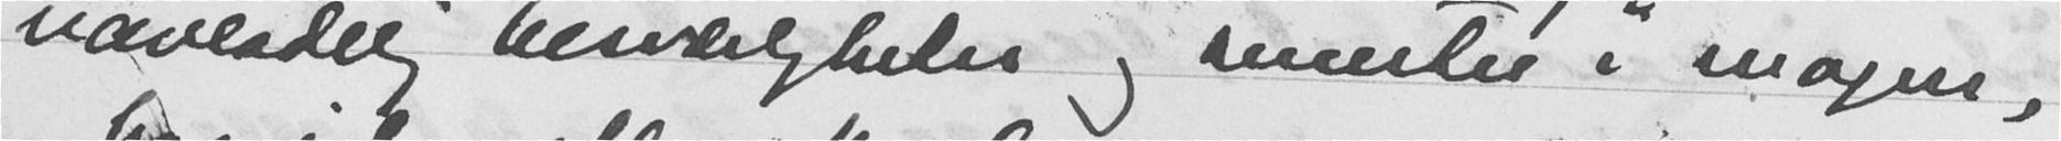uavladelig besværligheter og smerter i magen,
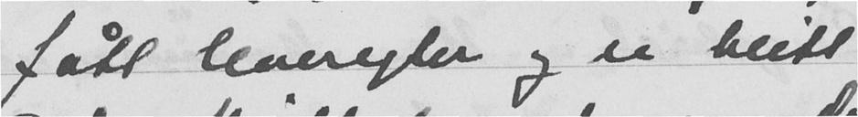fått leveregler og er blitt
